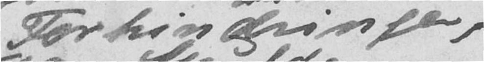Forhindringer,
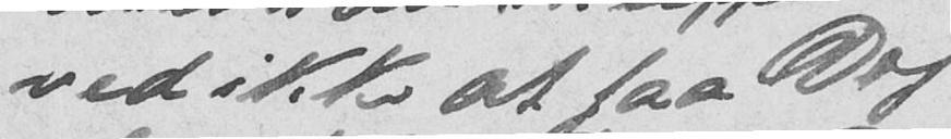ved ikke at faa Dig
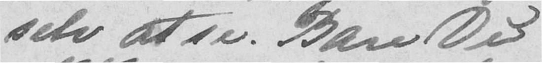selv at se. Bare Du
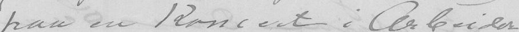paa en Koncert i Arbeider-
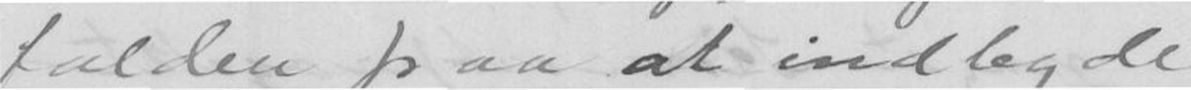falden paa at indlegde
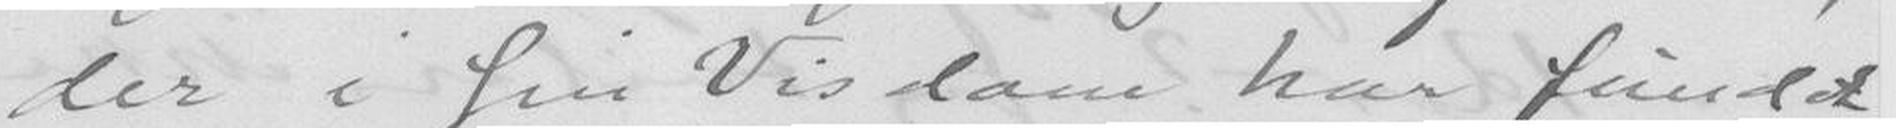der i sin Visdom har fundet
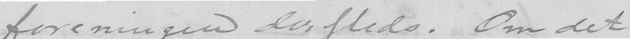foreningen dersteds. Om det
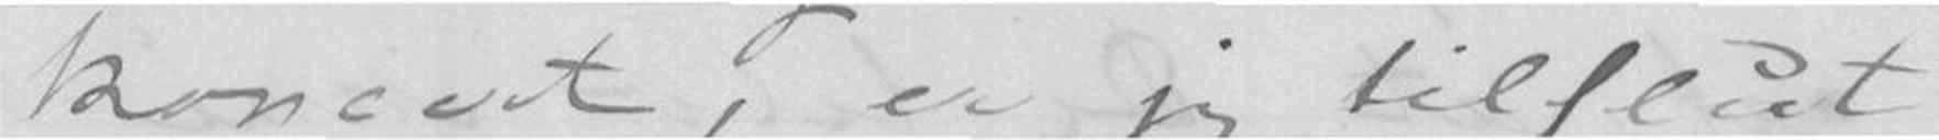koncert, er jeg tilslut
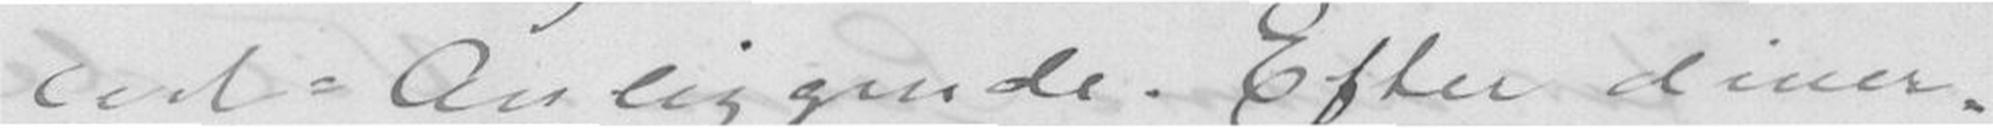cert=Anliggende. Efter diver-
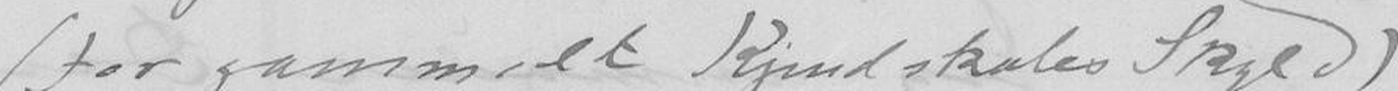(for gammelt Kjendskabs skyld)
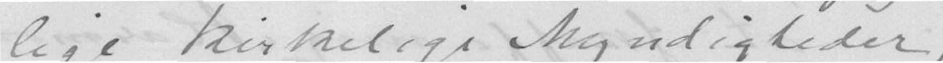lige kirkelige Myndigheder,
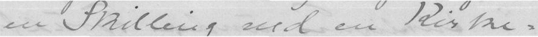en Skilling ved en Kirke-
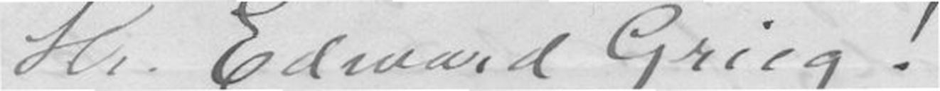Hr. Edward Grieg!
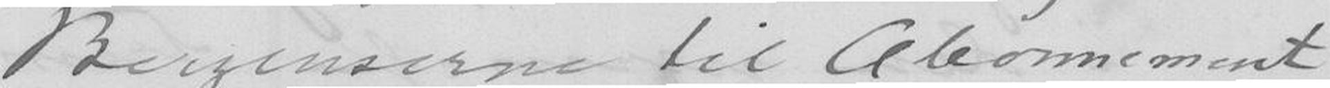Bergenserne til Abonnement
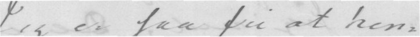Jeg er saa fri at hen-
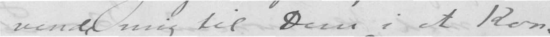vende mig til Dem i et Kon-
